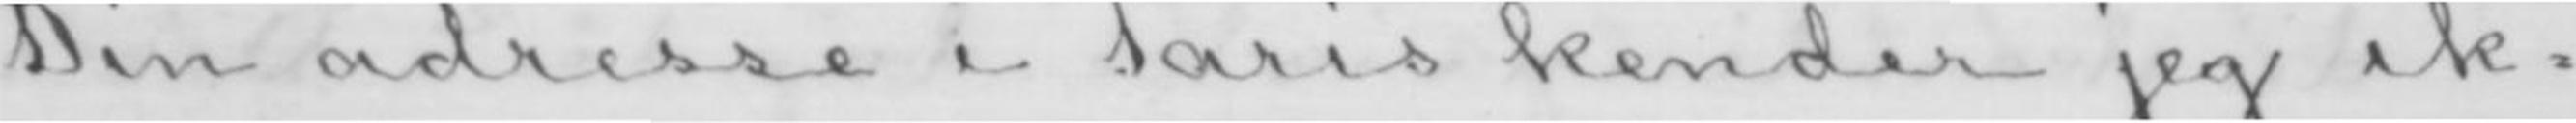Din adresse i Paris kender jeg ik-
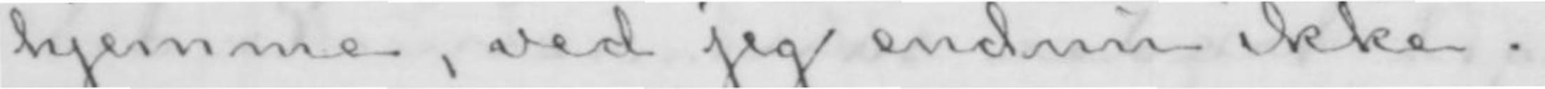hjemme, ved jeg endnu ikke.
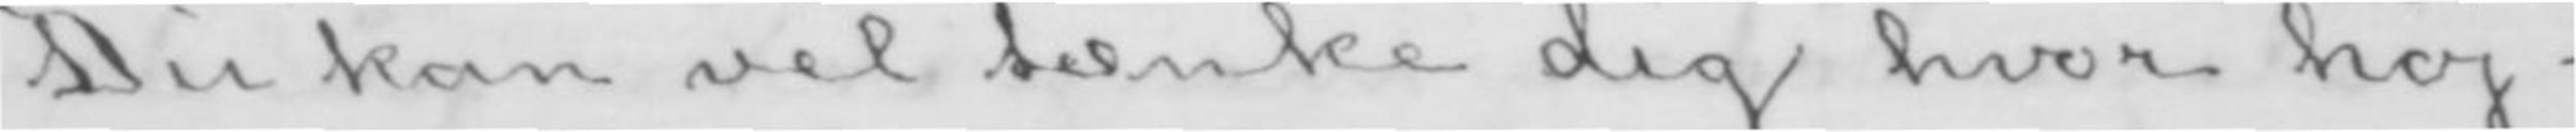Du kan vel tænke dig hvor høj-
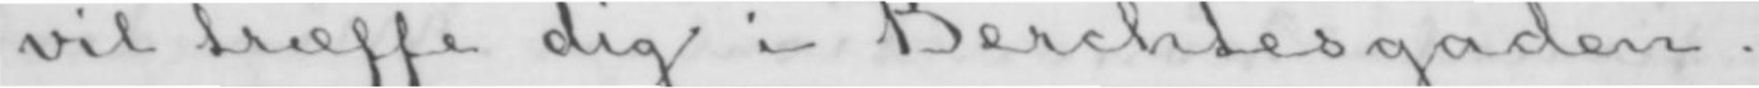vil træffe dig i Berchtesgaden.
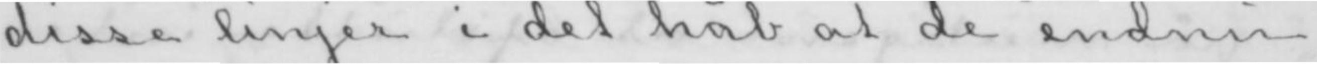disse linjer i det håb at de endnu
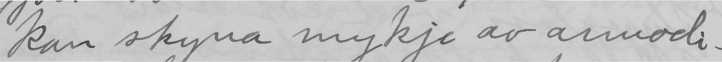kan skyna mykje av armodi.
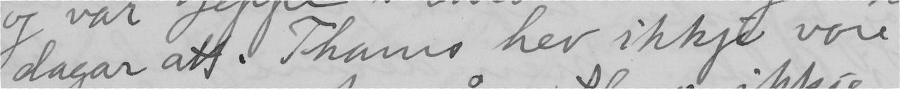dagar att. Thams hev ikkje vore
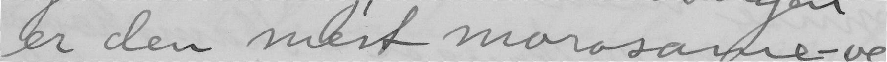er den mest morosame – og
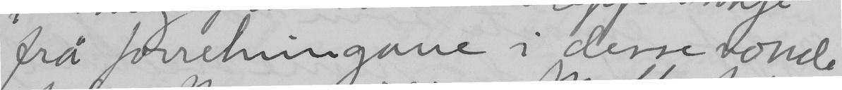frå forretningane i desse vonde
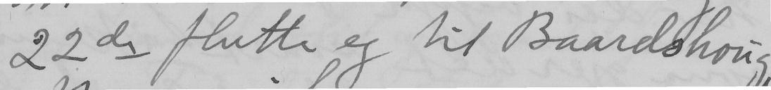22de flutte eg til Baardshoug
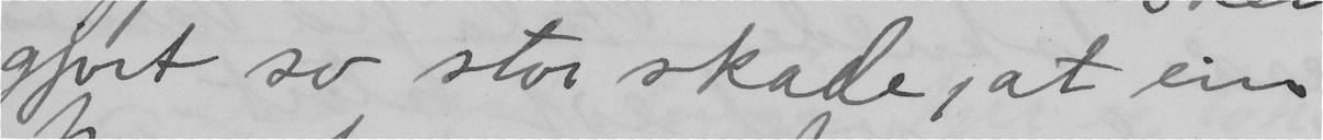gjort so stor skade, at ein
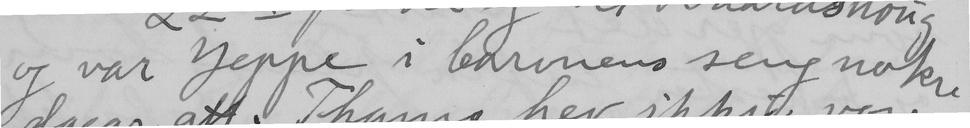og var Jeppe i baronens seng nokre
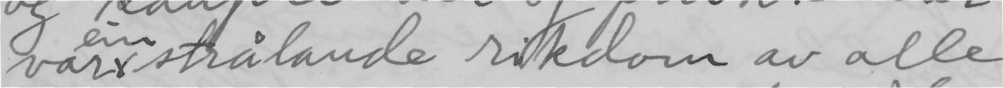var ein strålande rikdom av alle
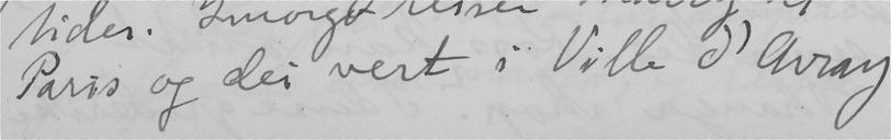Paris og dei vert i Ville d' Avray
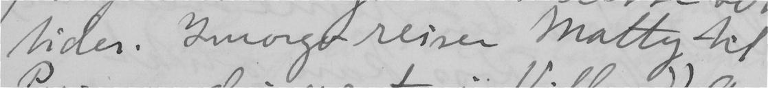tider. Imorgo reiser Matty til
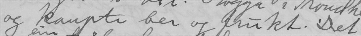og kaupte ber og frukt. Det
